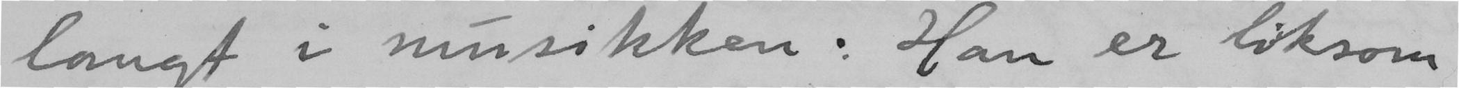langt i musikken. Han er liksom
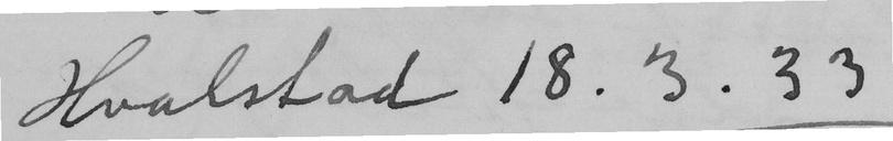Hvalstad 18.3.33
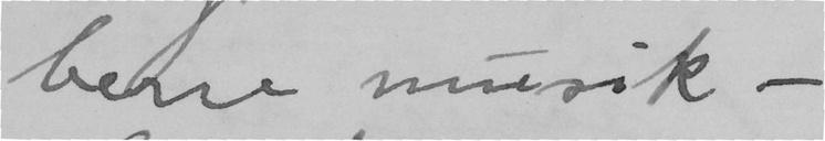berre musik –
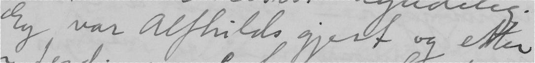Eg var Alfhilds gjest og etter
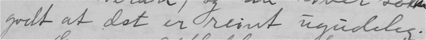godt at det er reint ugudeleg.
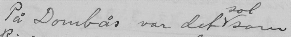På Dombås var det sol som
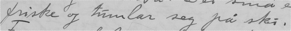friske og tumlar seg på ski.
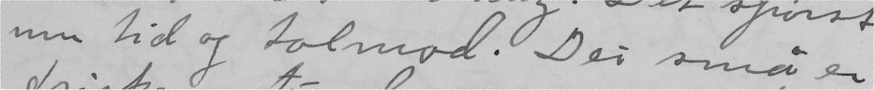um tid og tolmod. Dei små er
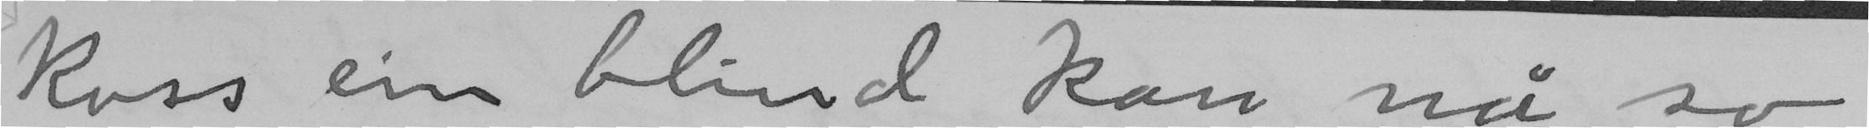koss ein blind kan nå so
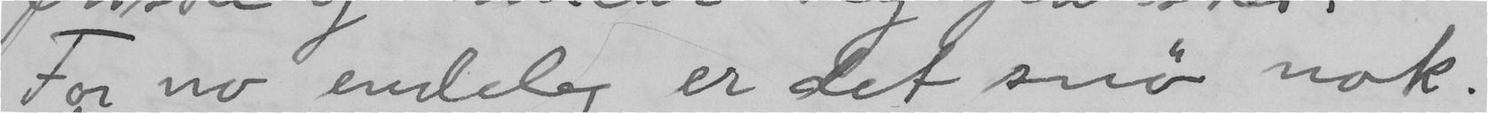For no endeleg er det sno nok.
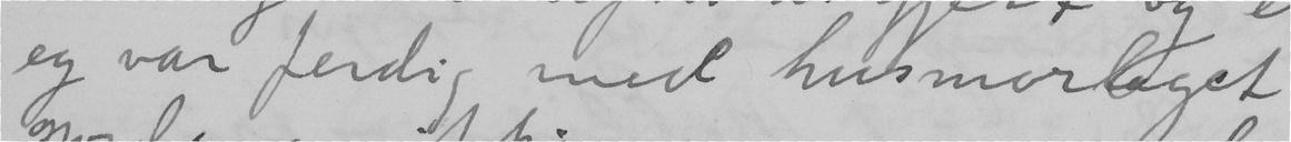eg var ferdig med husmorlaget
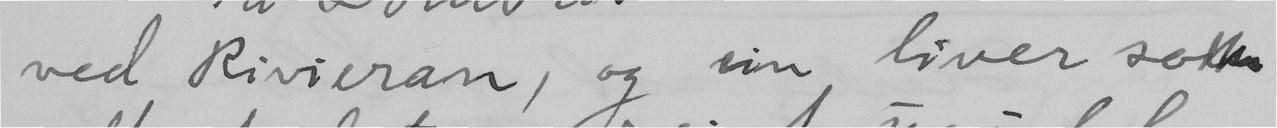ved Rivieran, og ein liver so
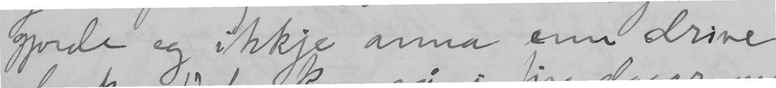gjorde eg ikkje anna enn drive
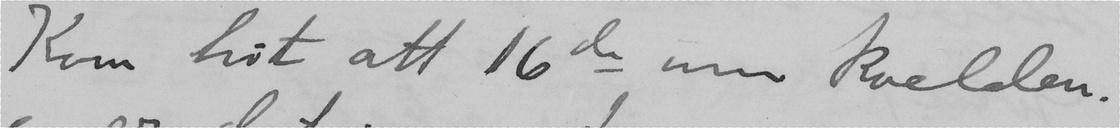Kom hit att 16de um kvelden.
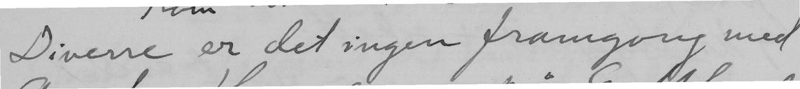Diverre er det ingen framgong med
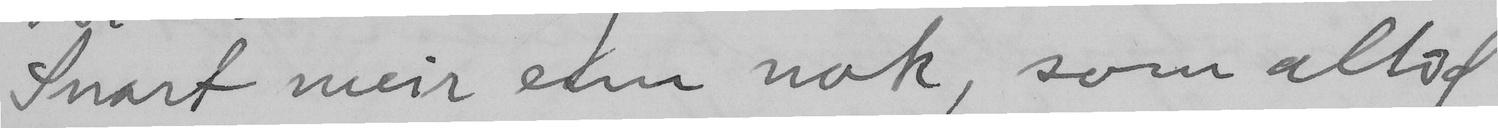Snart meir enn nok, som altid
